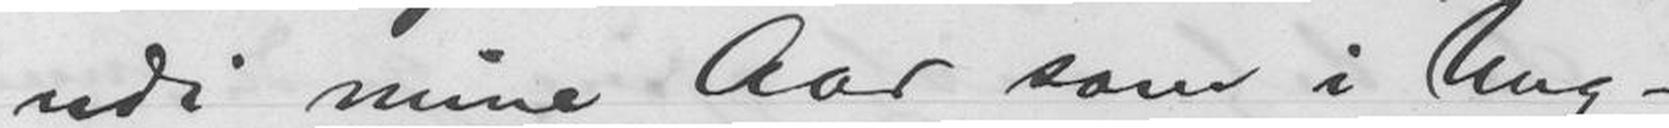udi mine Aar som i Ung-
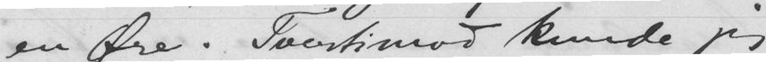en Øre. Tvertimod kunde jeg
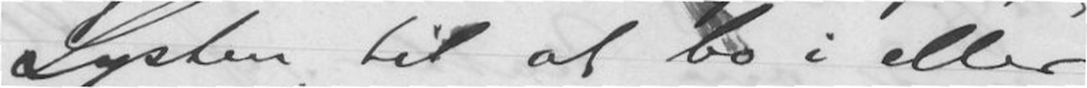Lysten til at bo i eller
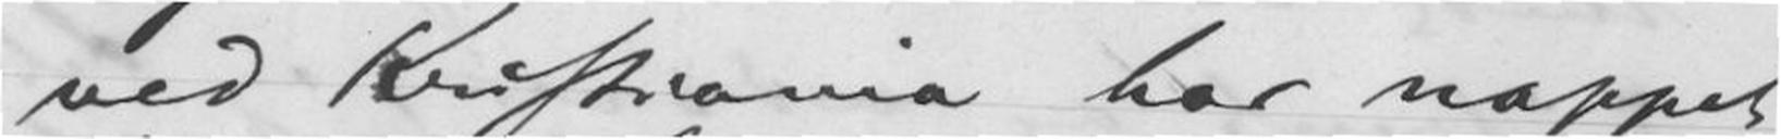ved Kristiania har nappet
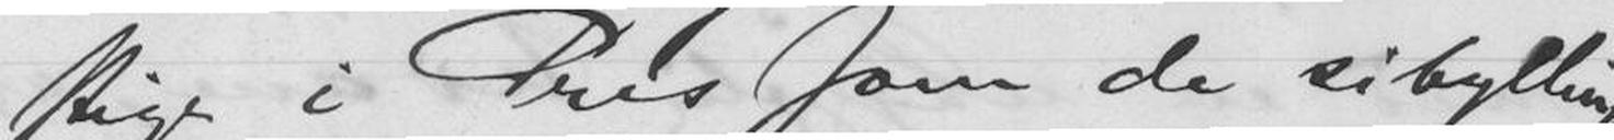det stige i Pris som de sibyllinske
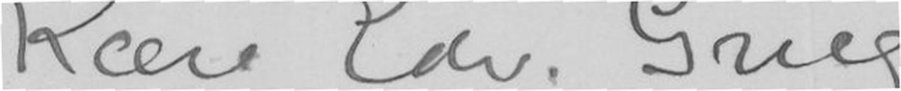Kære Edv. Grieg!
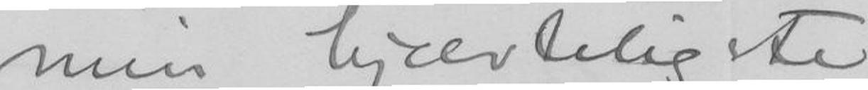min hjærteligste
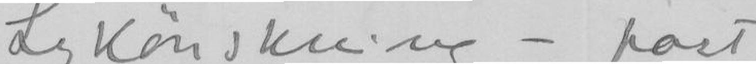Lykønskning - post
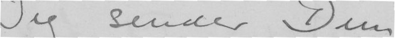Jeg sender Dem
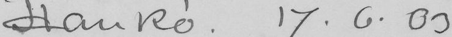Hankø. 17.6.03
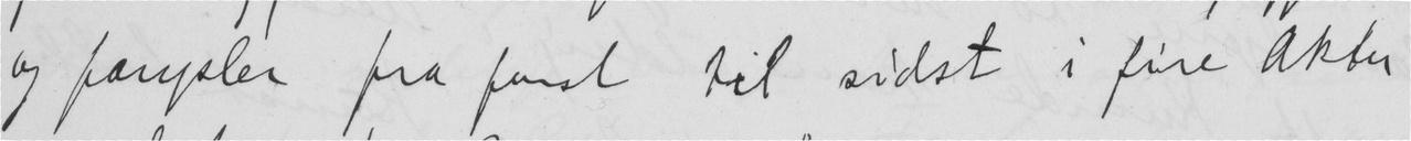og fængsler fra først til sidst i fire Akter
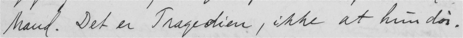Mand. Det er Tragedien, ikke at hun dør.
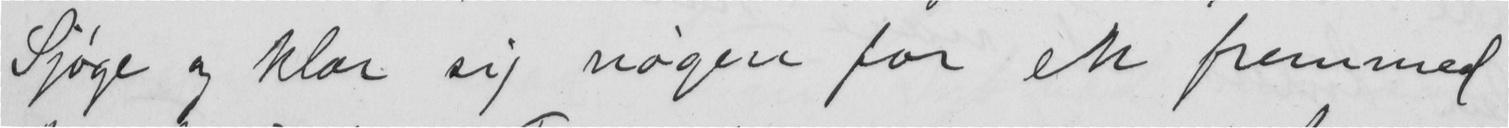Sjøge og klær sig nøgen for en fremmed
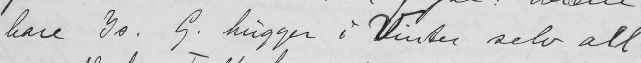bare Is. G. hugger i Vinter selv alt
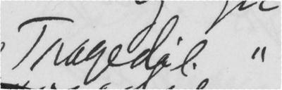Tragedie"
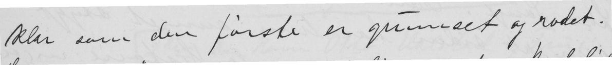klar som den første er grumset og rodet?
